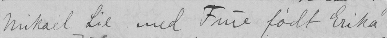Mikael Lie med Frue født Erika
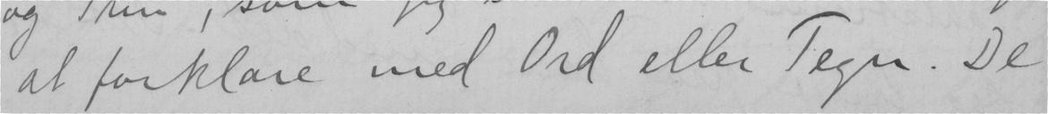at forklare med Ord eller Tegn. De
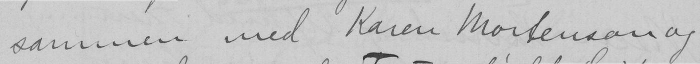sammen med Karen Mortenson og
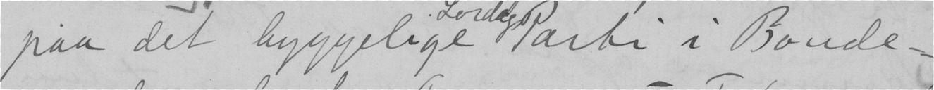paa det hyggelige Parti i Bonde-
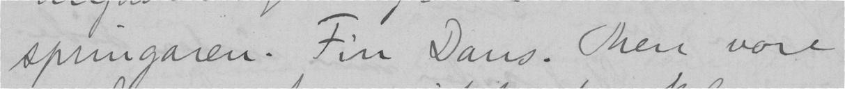springaren. Fin Dans. Men vore
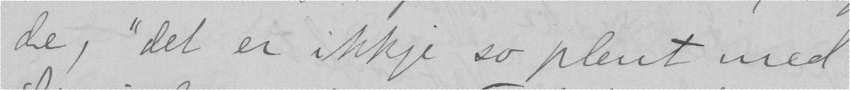de, "det er ikkje so plent med
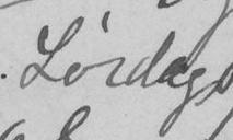Lørdag
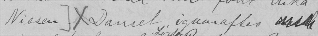Nissen. x Danset igaaraftes
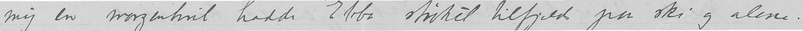tok mig en morgenhvil hadde Ebba strøket tilfjelds paa ski og alene.
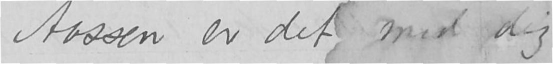Aassen er det med dig? Nu er det længe siden jeg
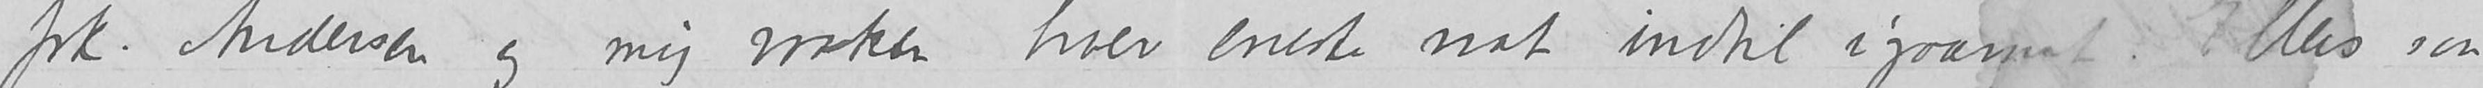frk. Andersen og mig vaaken hver eneste nat indtil igaarnat. Ellers saa
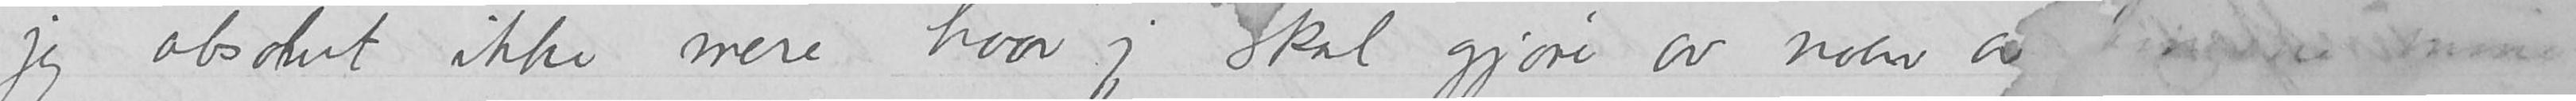jeg absolut ikke mere hvor jeg skal gjøre av noen av tingene mine
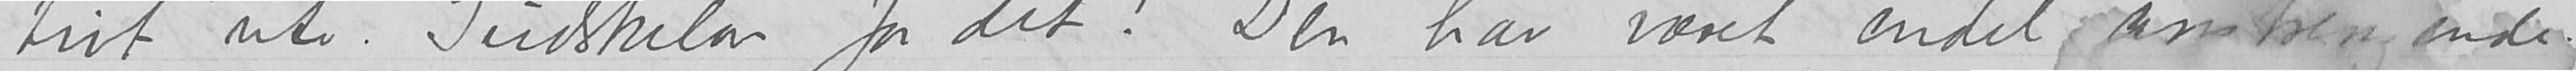tivt ute. Gudskelov for det! Den har været en del anstrengende.
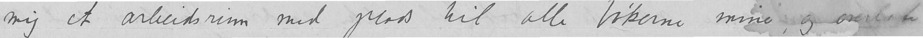faa mig et arbeidsrum med pladss til alle bøkerne mine, og overlate
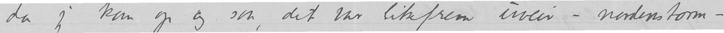da jeg kom op og saa, det var likefrem uveir – nordenstorm –
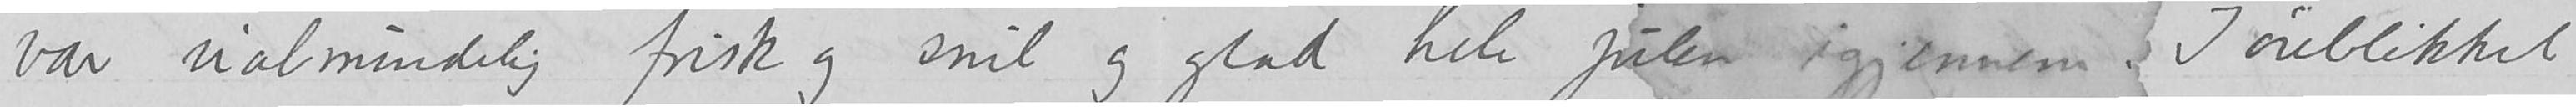var ualmindelig frisk og snil og glad hele julen igjennem. I øieblikket
